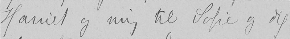Harriet og mig til Sofie og dig
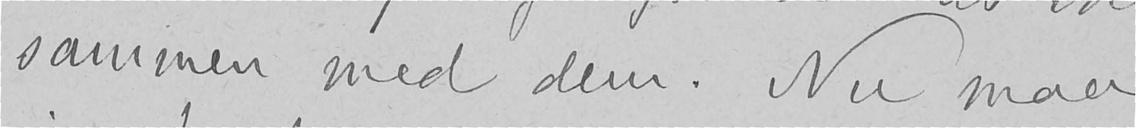sammen med dem. Nu maa
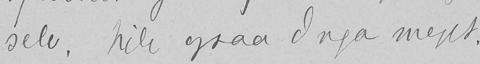selv, hils ogsaa Inga meget,
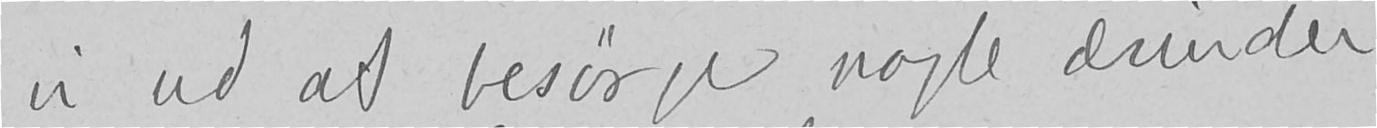vi ud at besørge nogle ærinder,
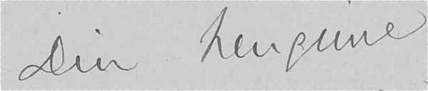Din hengivne
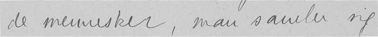de mennesker, man samle sig
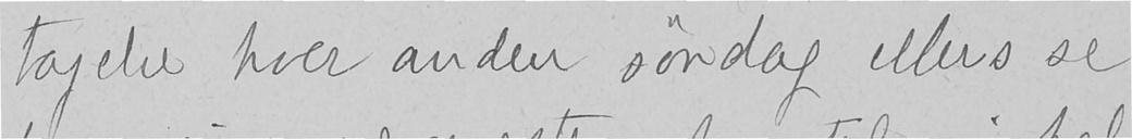tagelse hver anden søndag, ellers se
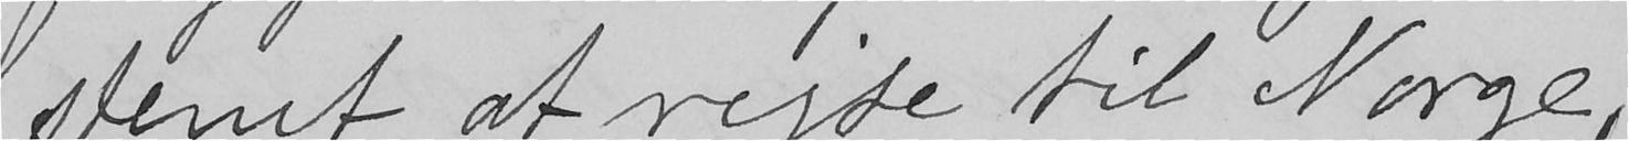stemt at rejse til Norge,
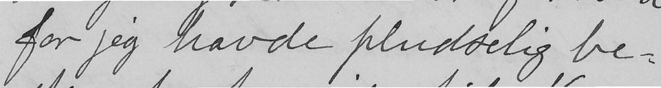for jeg havde pludselig be-
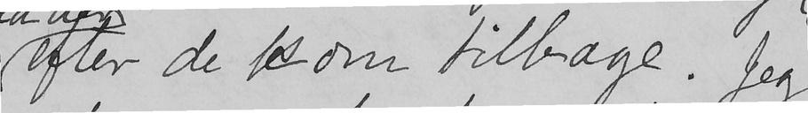efter de kom tilbage. Jeg
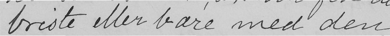briste eller bære med den
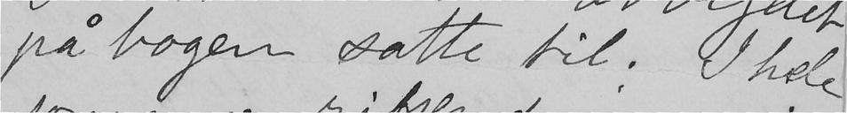på bogen satte til. I hele
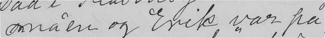småen og Erik var på
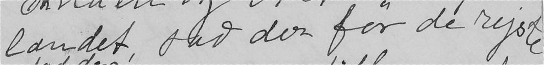landet, sad der før de rejste
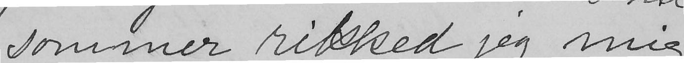sommer rikked jeg mig
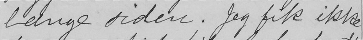længe siden. Jeg fik ikke
